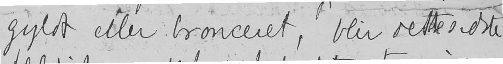gyldt eller bronceret, blir dette sidste
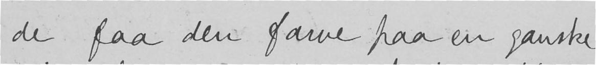de faa den farve paa en ganske
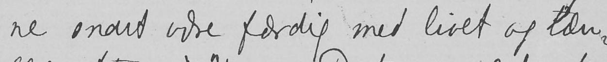ne snart være færdig med livet og læn-
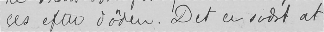ges efter døden. Det er svært at
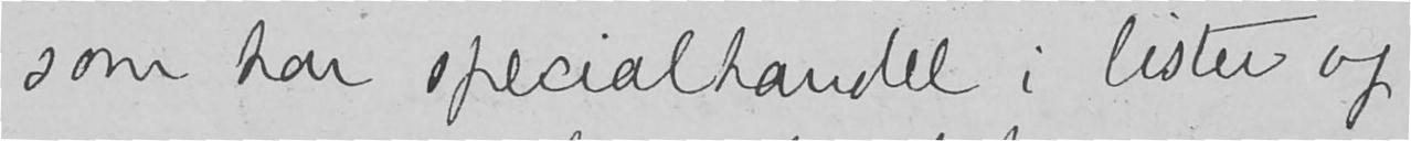som har specialhandel i lister og
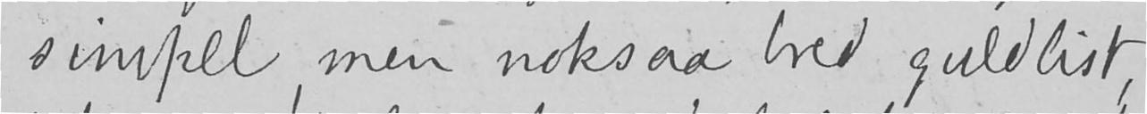simpel, men noksaa bred guldlist,
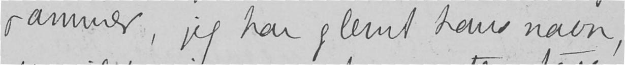rammer, jeg har glemt hans navn,
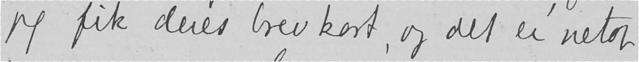jeg fik deres brevkort, og det er netop
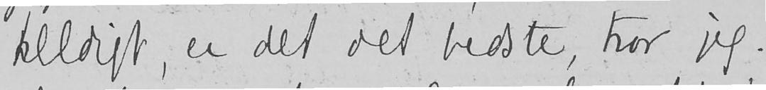heldigt, er det det bedste, tror jeg.
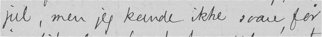jul, men jeg kunde ikke svare, før
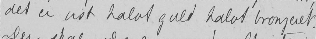det er vist halvt guld halvt bronzeret.
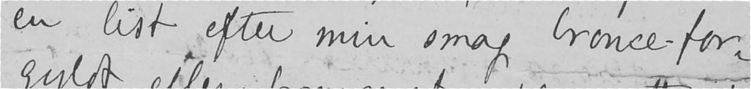en list efter min smag bronce-for-
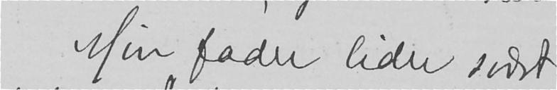- Min fader lider svært
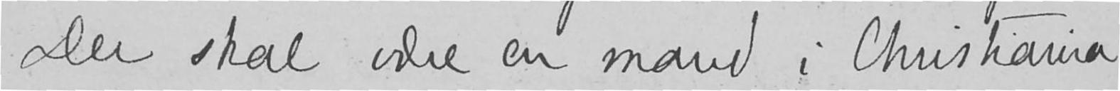Der skal være en mand i Christiania
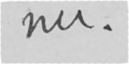nu.
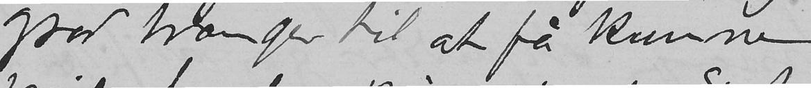grad trænger til at få kunne
